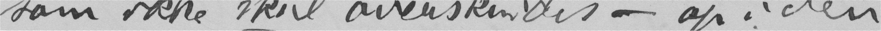som ikke skal overskrides – op i den
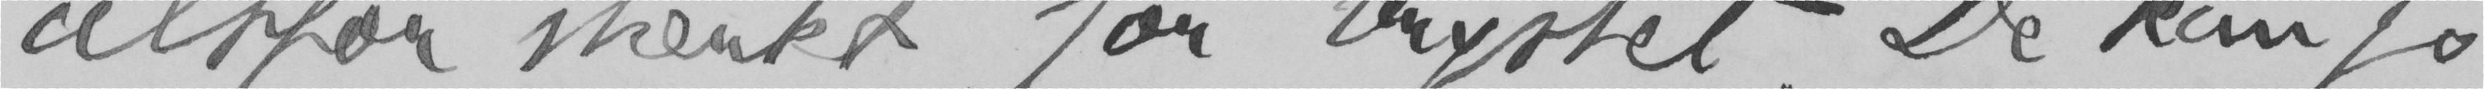altfor stærkt for brystet. De kan jo
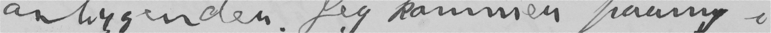anliggender. Jeg kommer paany i
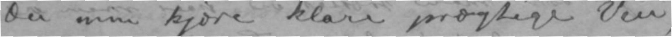Du min kjære klare prægtige Ven,
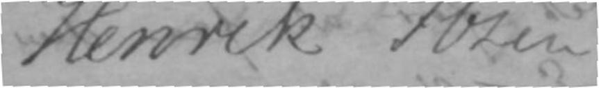Henrik Ibsen
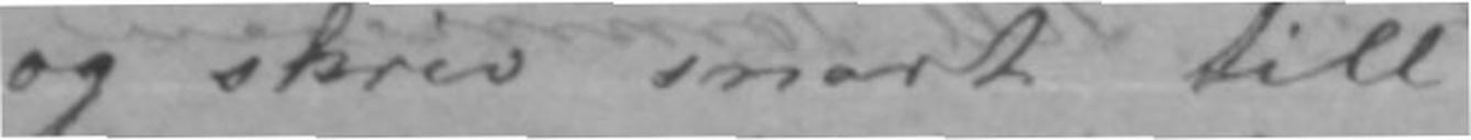og skriv snart till
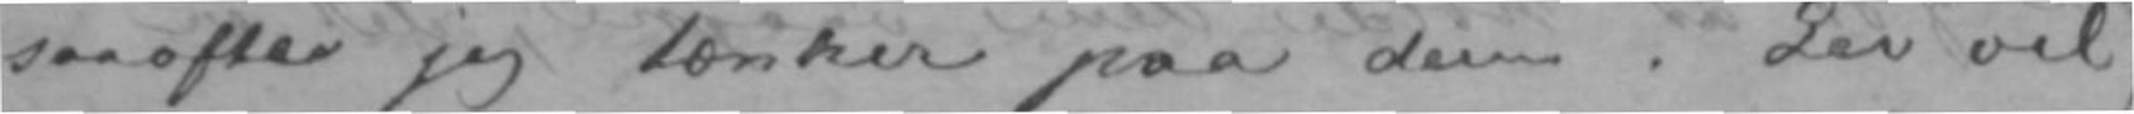saaofte jeg tænker paa dem. Lev vel,
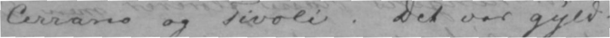Cerrano og Tivoli. Det var gyld-
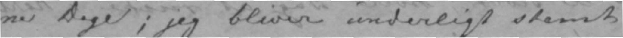ne Dage; jeg bliver underligt stemt
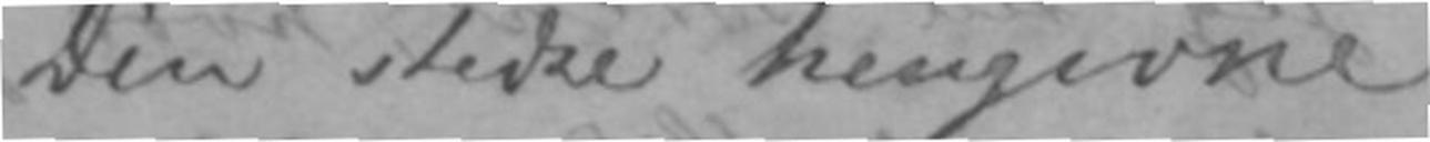Din stedse hengivne
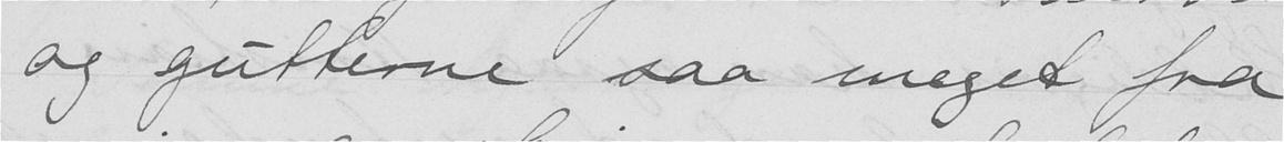og gutterne saa meget fra
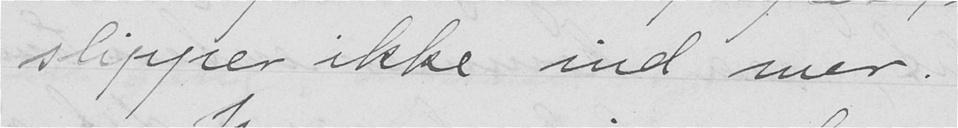slipper ikke ind mer.
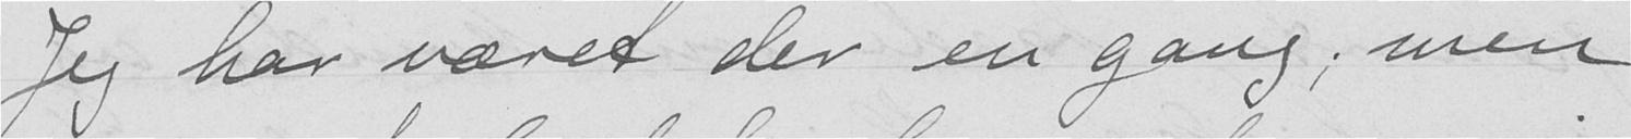Jeg har været der en gang; men
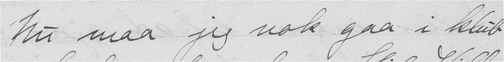Nu maa jeg nok gaa i klub-
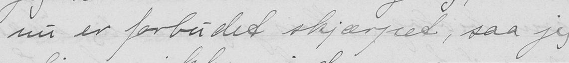nu er forbudet skjærpet, saa jeg
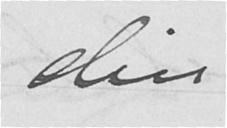din
